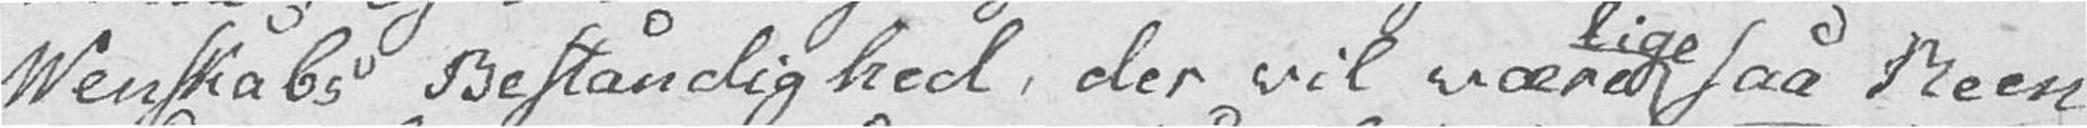Venskabs Bestandighed, der vil være lige saa Reen
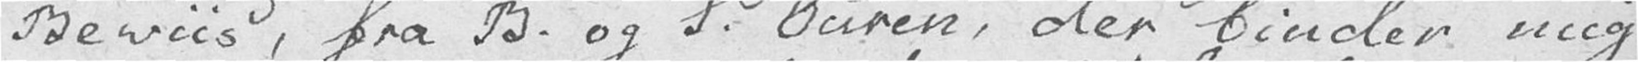Beviis, fra B. og S. Ouren, der binder mig
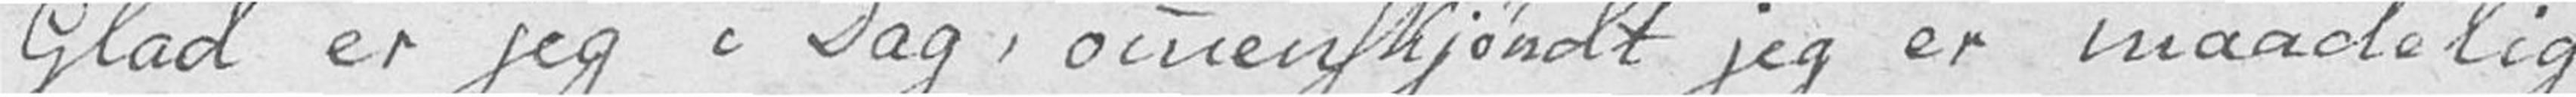Glad er jeg i Dag, omenskjøndt jeg er maadelig
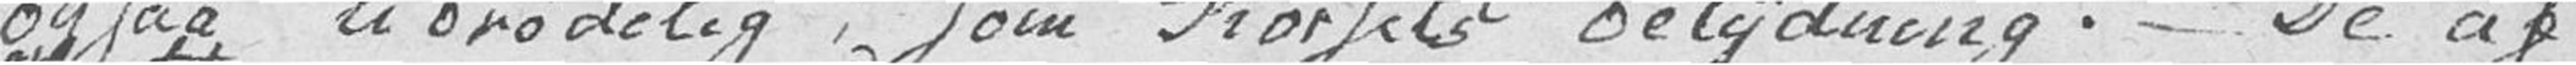ogsaa ubrødelig, som Korsets betydning. - De af
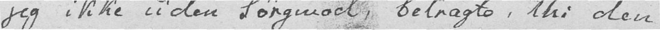jeg ikke uden Sørgmod betragte, thi den
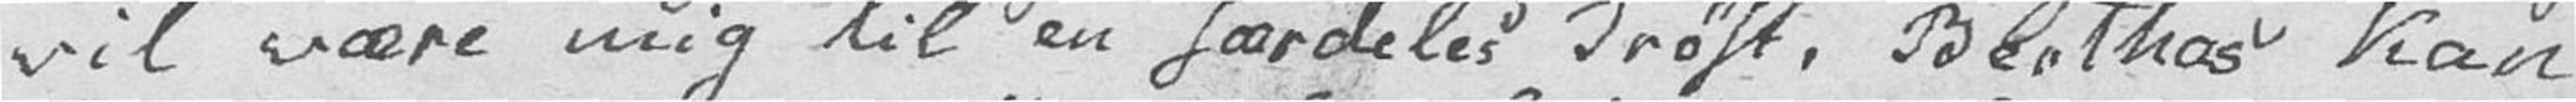vil være mig til en særdeles Trøst, Berthas kan
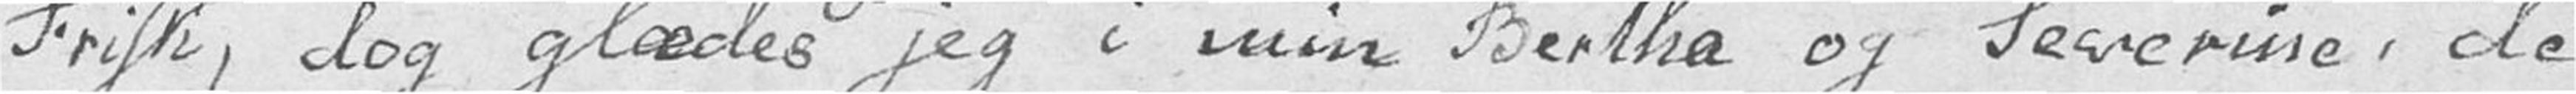Frisk, dog glædes jeg i min Bertha og Severine, de
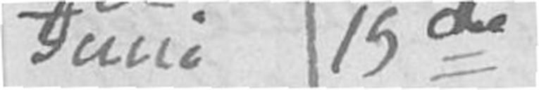Juni 15de
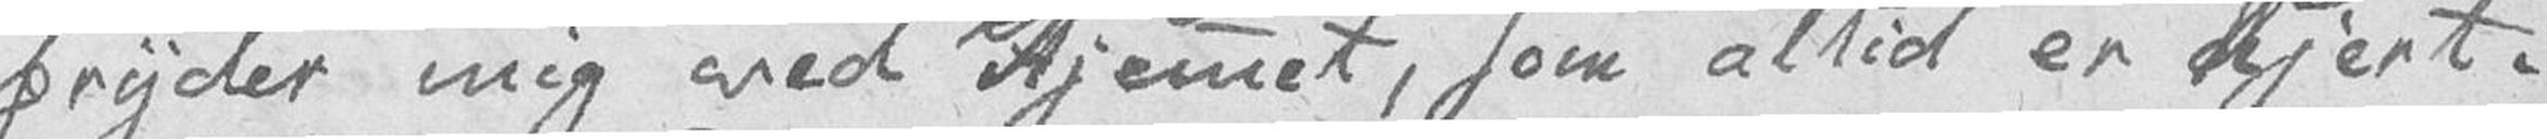fryder mig ved Hjemmet, som altid er Kjert.
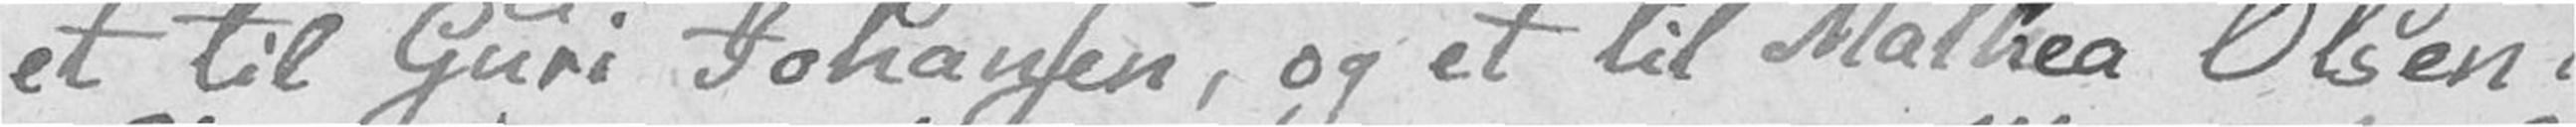et til Guri Johansen, og et til Mathea Olsen i
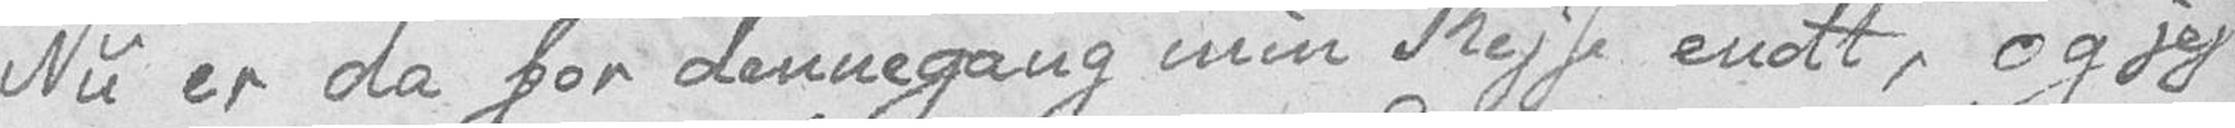Nu er da for dennegang min Rejse endt, og jeg
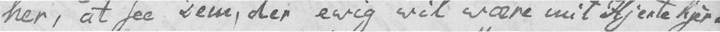her, at see Dem, der evig vil være mit Hjerte kjer.
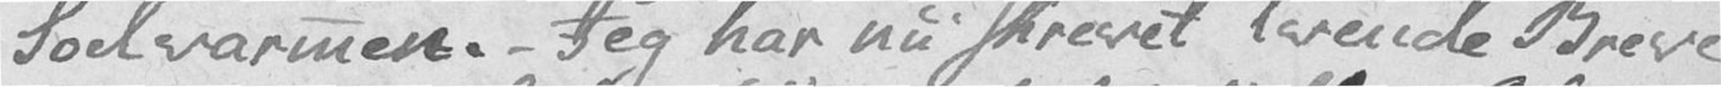Soelvarmen. – Jeg har nu skrevet tvende Breve
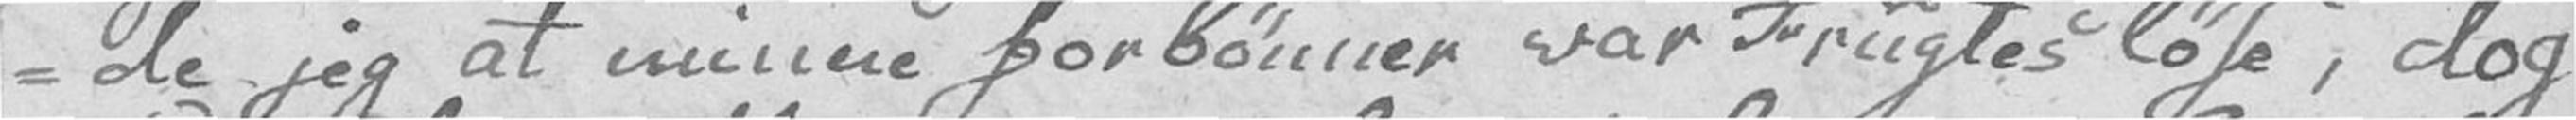-de jeg at minne forbønner var Frugtesløse, dog
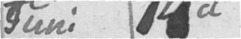Juni 14d
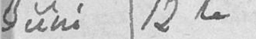Juni 12te
